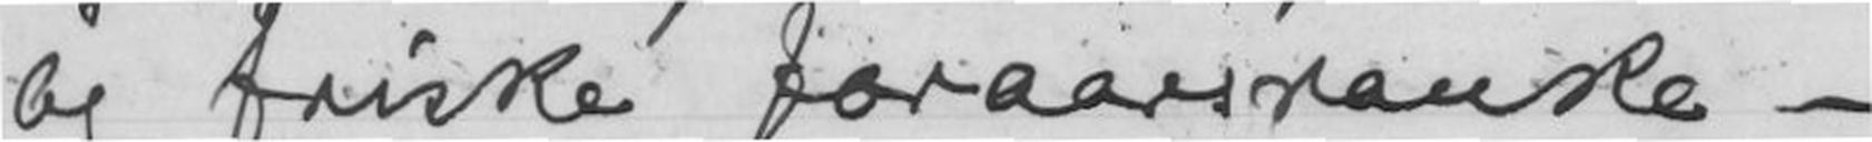og friske foraarsranke -
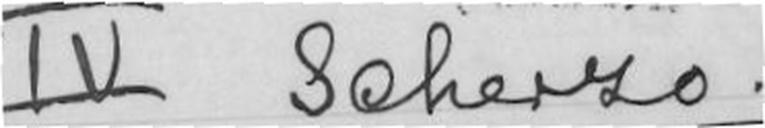IV Scherzo.
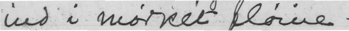ind i mørket fløine -
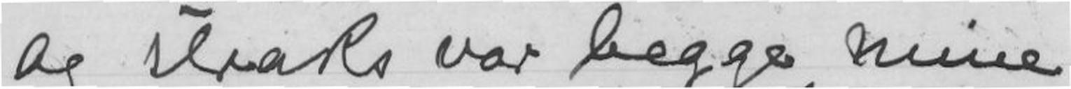og straks var begge mine
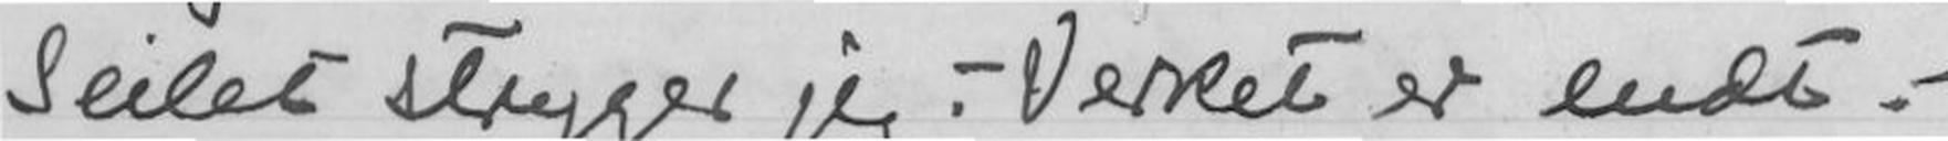Seilet stryger jeg. - Verket er endt. -
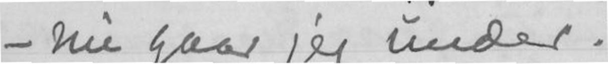- nu gaar jeg under!
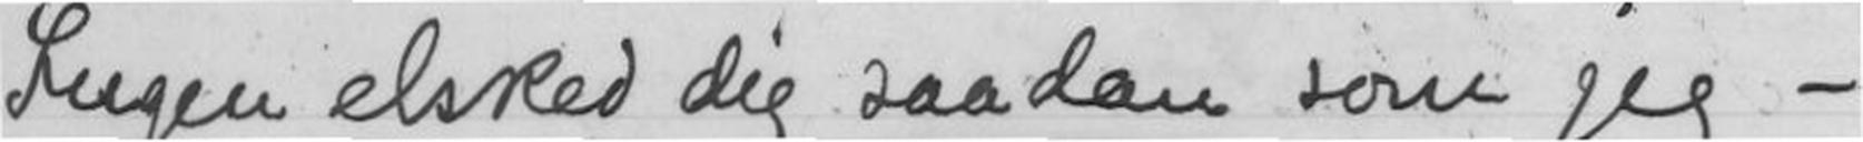Ingen elsked dig saadan som jeg -
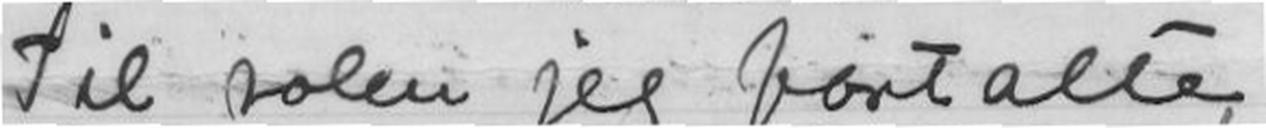Til solen jeg fortalte,
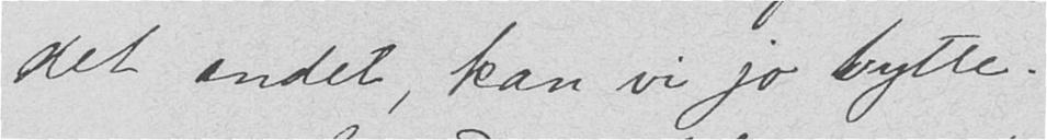det andet, kan vi jo bytte.
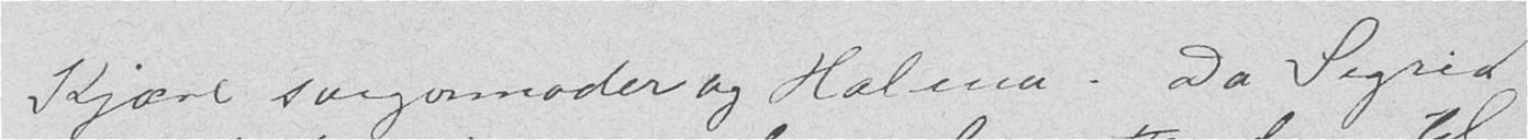Kjære svigermoder og Halma? Da Sigrid
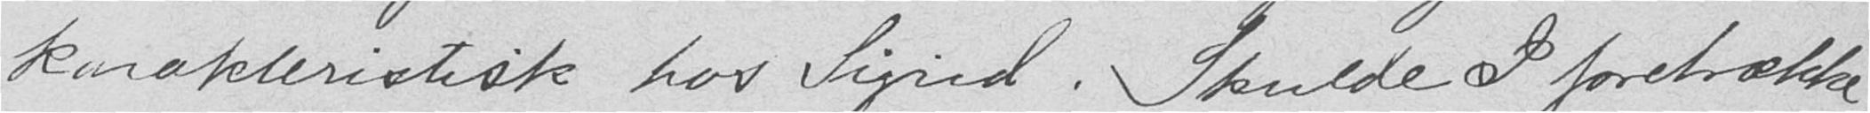karakteristisk hos Sigrid. Skulde I foretrække
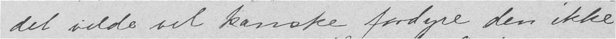det vilde vel kanske fordyre den ikke
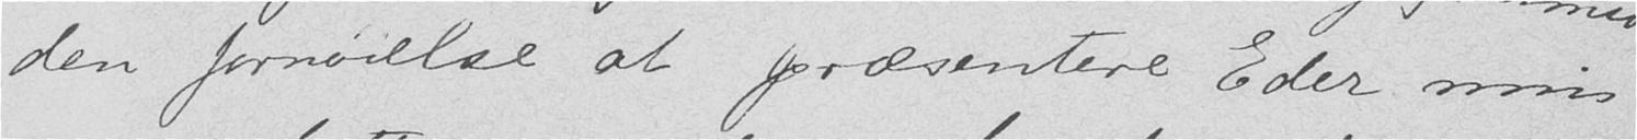den fornøielse at præsentere Eder min
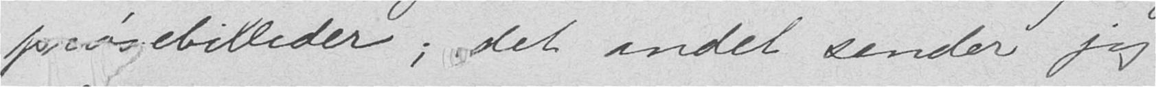prøvebilleder; det andet sender jeg
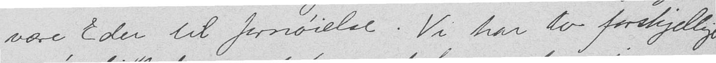være Eder til fornøielse. Vi har to forskjellige
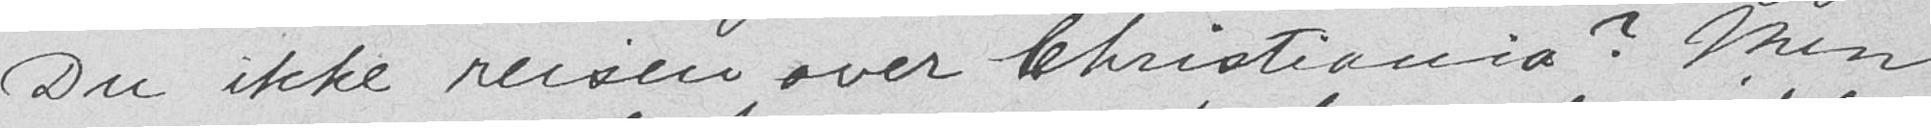Du ikke reisen over Christiania? Men
